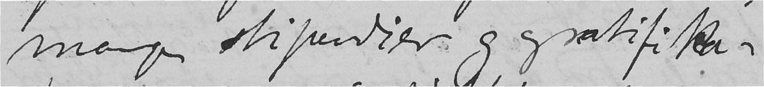mange stipudier og gratifika-
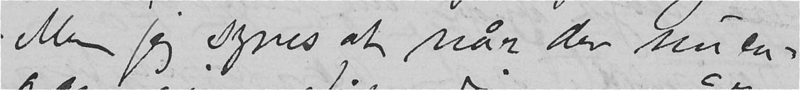eller jeg synes at når der nu en-
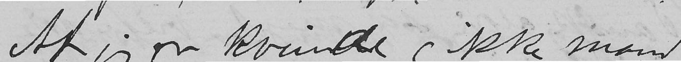At jeg er kvinde og  ikke mand
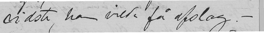vidste, han vilde få afslag.-
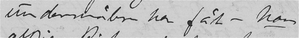undermålere har fåt - han
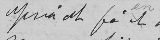opnå at få et
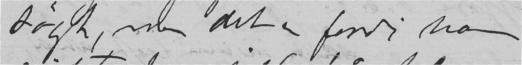søgt, men det er fordi han
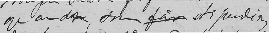ge andre som får stipendier,
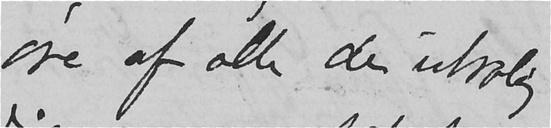øre af alle de utrolig
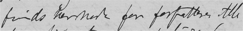finds hernede for forfattere. Alle
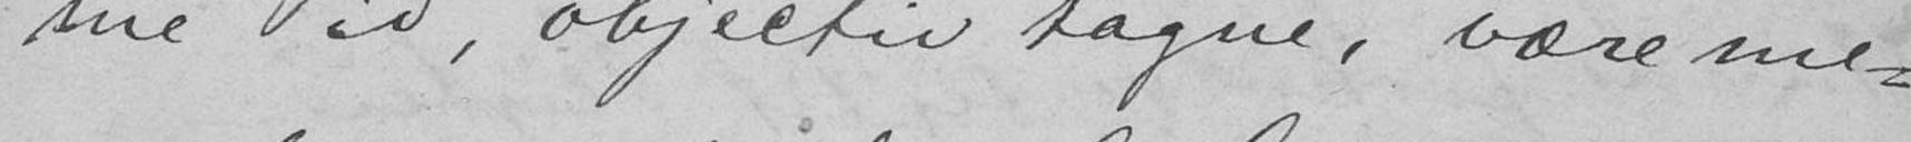me Tid, objectiv tagne, være me-
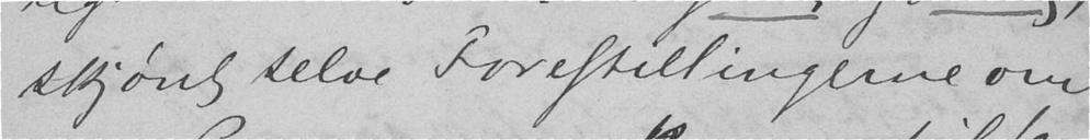skjønt selve Forestillingerne om
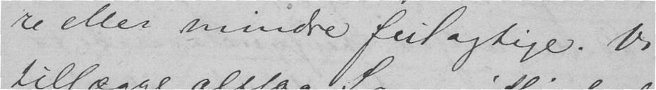re eller mindre feilagtige. Vi
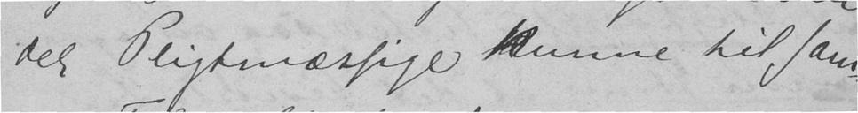del Pligtmæssige kunne til sam-
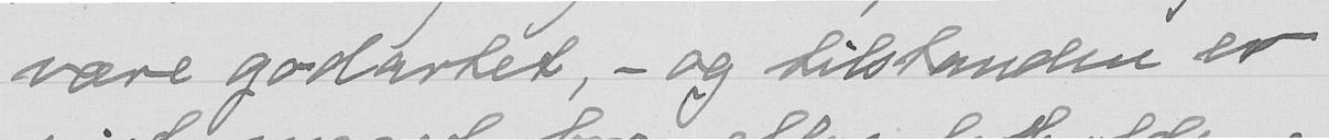være godartet, - og tilstanden er
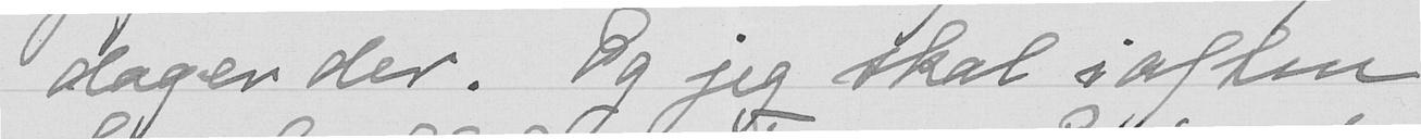dager der. Og jeg skal i aften
